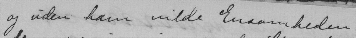og uden ham vilde Ensomheden
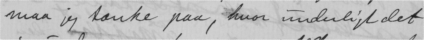maa jeg tænke paa, hvor underligt det
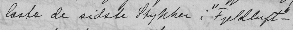læste de sidste Stykker i "Fjeldluft" -
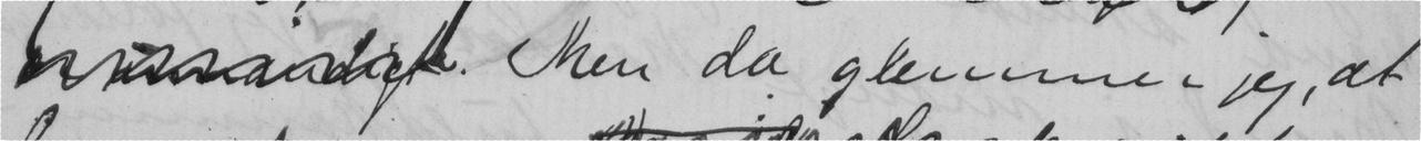. Men da glemmer jeg, at
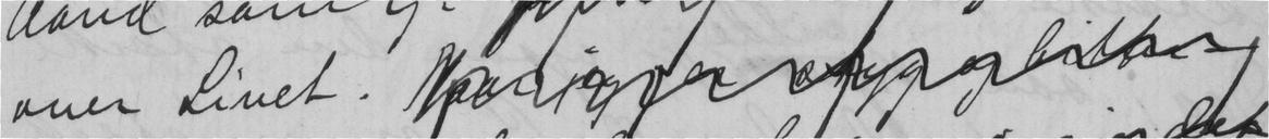over Livet.
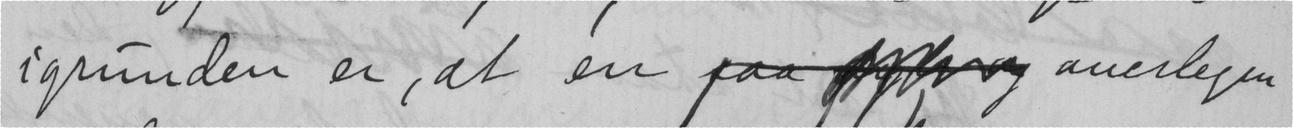i grunden er, at en saa  overlegen
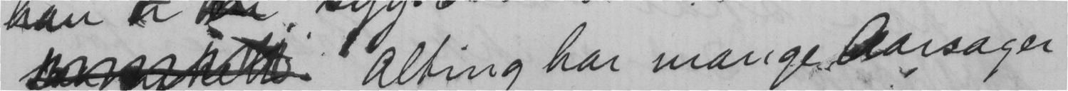Alting har mange Aarsager
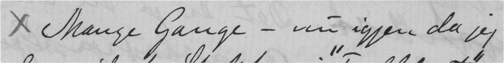x Mange Gange - nu igjen da jeg
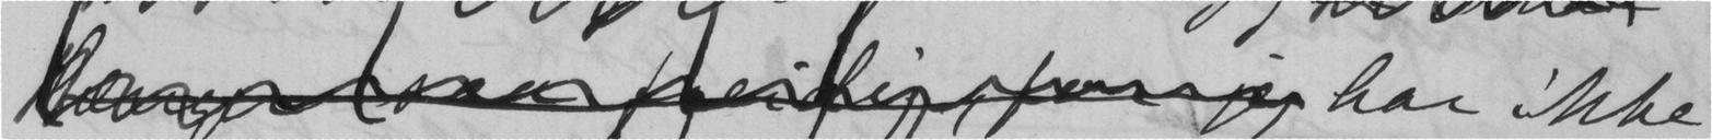har ikke
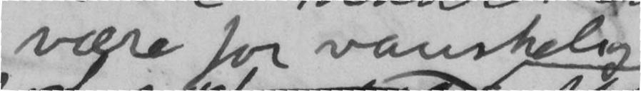være for vanskelig
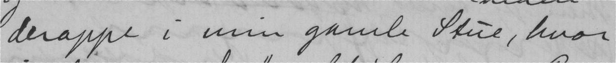deroppe i min gamle Stue, hvor
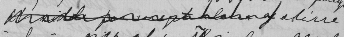stirre
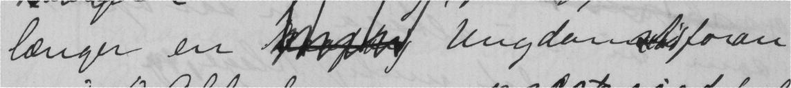længer en  Ungdomstid foran
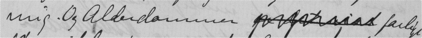mig. Og Alderdommen  farligt
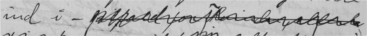ind i -
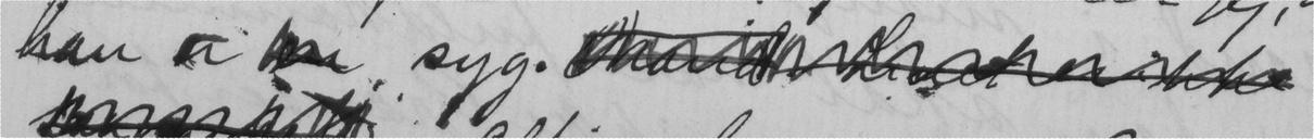han er en syg.
